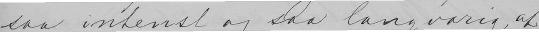saa intenst og saa langvarig, at
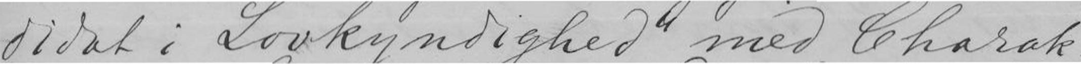didat i Lovkyndighed med Charak
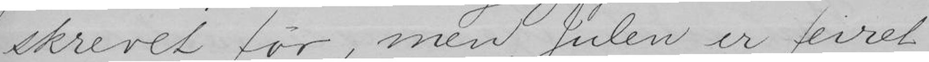skrevet før, men Julen er feiret
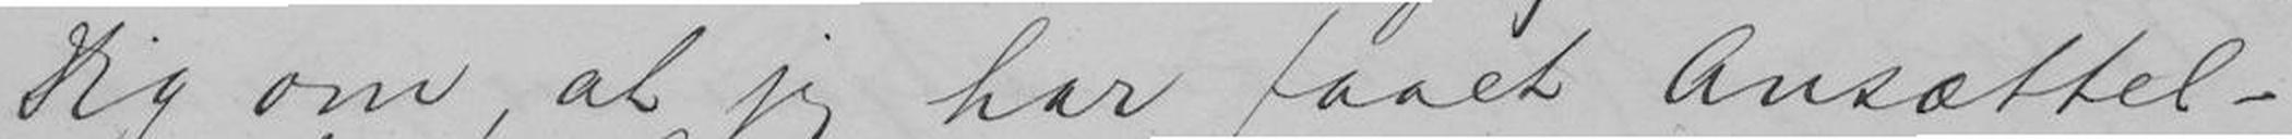Dig om, at jeg har faaet Ansættel-
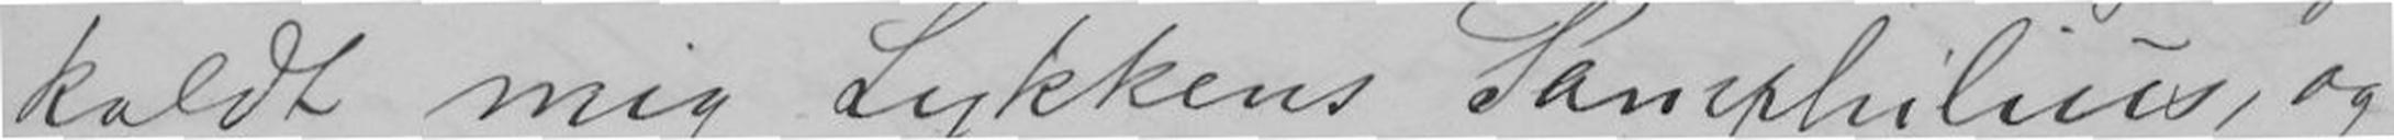kaldt mig Lykkens Pamphilius, og
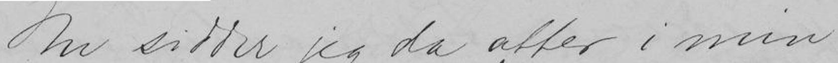Nu sidder jeg da atter i min
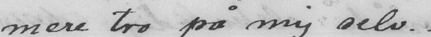mere tro på mig selv. -
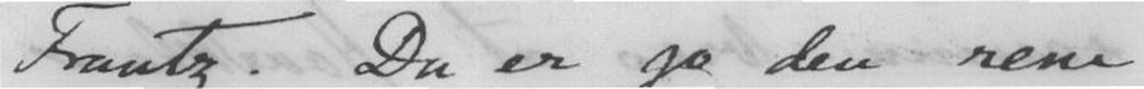Frantz. Du er jo den rene
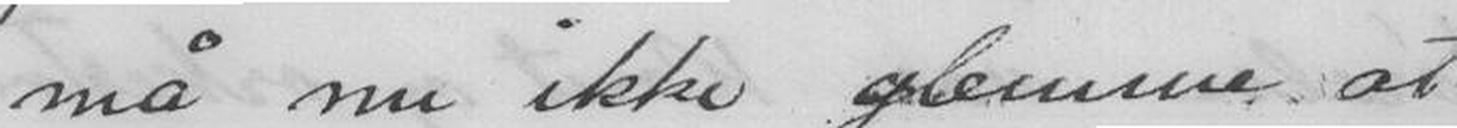må nu ikke glemme at
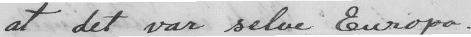at det var selve Europa.
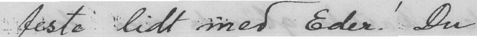feste lidt med Eder! Du
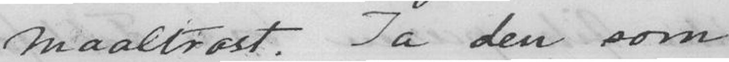maaltrost. Ja den som
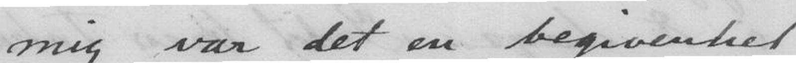mig var det en begivenhed
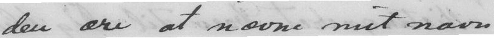den ære at nævne mit navn
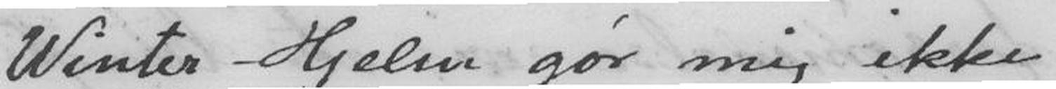Winter-Hjelm gør mig ikke
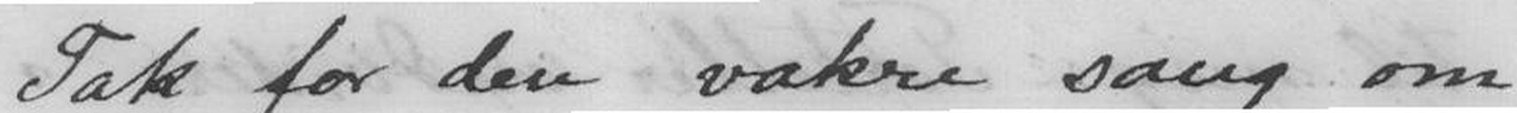Tak for den vakre sang om
